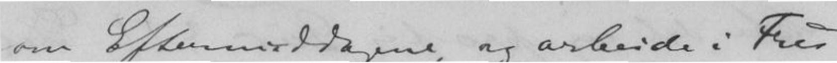om Eftermiddagene og Arbeide i Fred
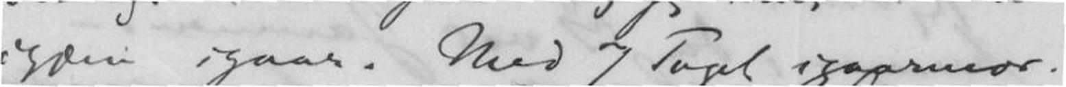igjen igaar. Med 7 Toget igaarmor-
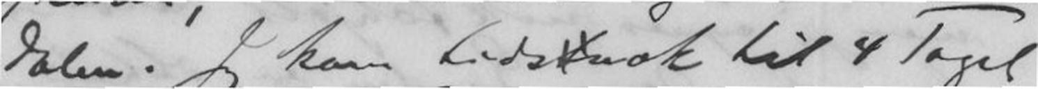dalen. Jeg kom tidsnok til 4 Toget
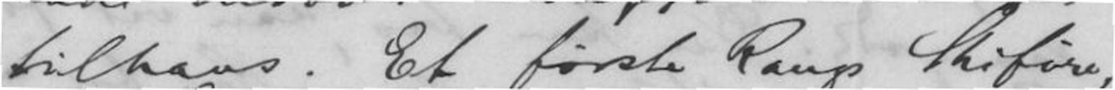tilhavs. Et første Rangs Skiføre,
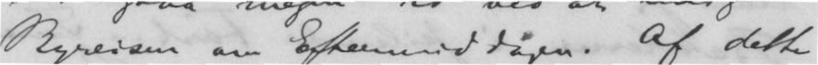Byreisen om Eftermiddagen. Af dette
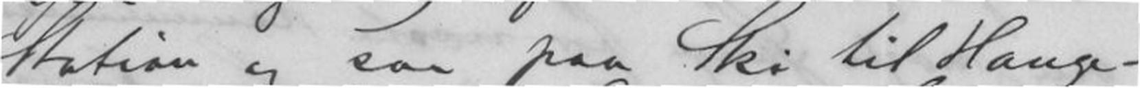Station og saa paa Ski til Hauge-
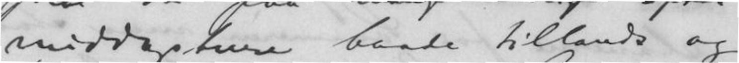middagsture baade tillands og
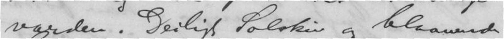varden. Deiligt Solskin og blaanende
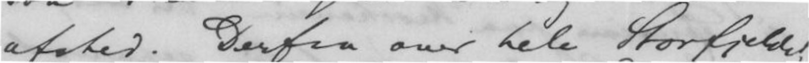afsted. Derfra over hele Storfjeldet,
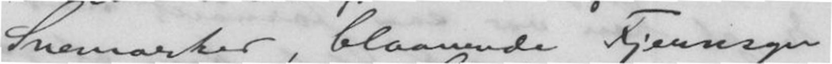Snemarker, blaanende Fjernsyn
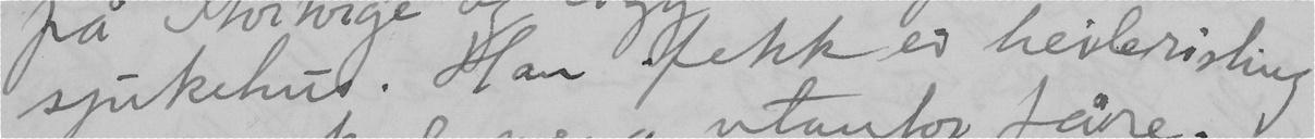sjukehus. Han fekk ei heuderistung
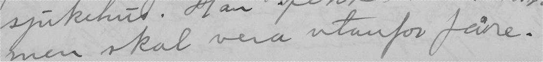men skal vera utanfor fåre.
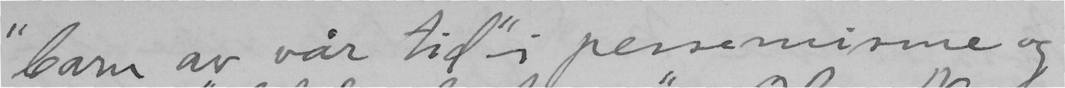"barn av vår tid" i pessimisme og
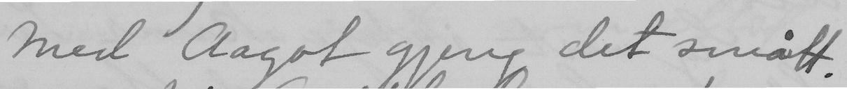Med Aagot gjeng det smått;
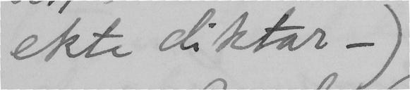ekte diktar –)
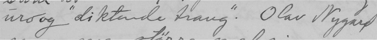uro og "diktande trang". Olav Nygard
